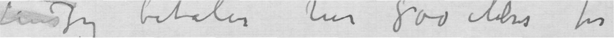Lins Jeg betaler her 800 Mks for
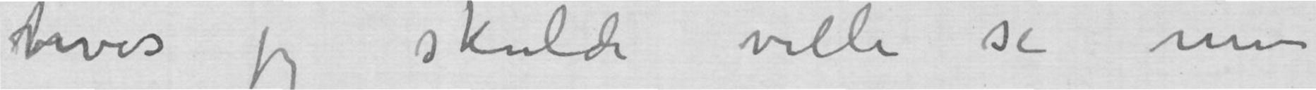hvis jeg skulde ville se min
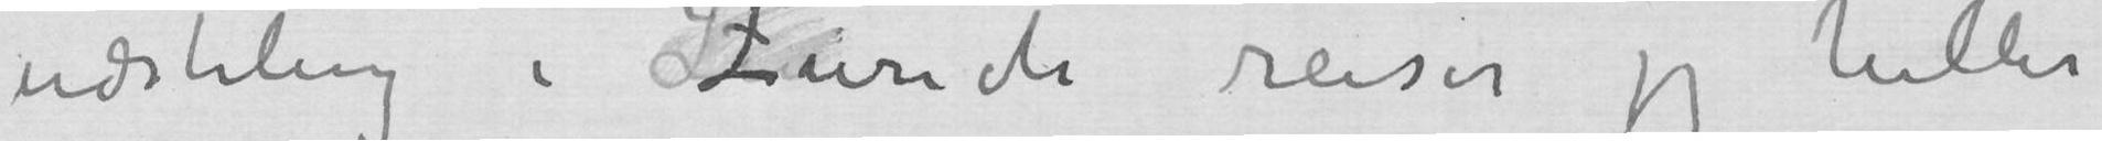udstilling i Zürich reiser jeg heller
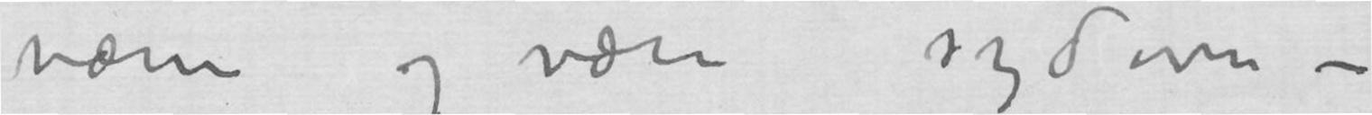værre og værre sydover –
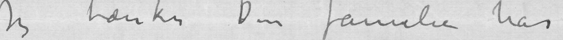Jeg tænker Din familie har
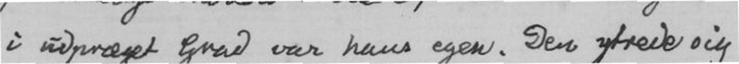i udpræget grad var hans egen. Den ytrede sig
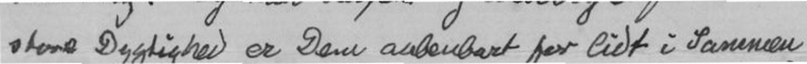store Dygtighed er Dem aabenbart for lidt i Sammæn-
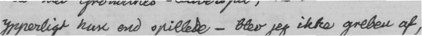ypperligt hun end spillede - blev jeg ikke greben af,
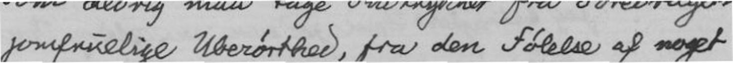Jomfruelige Uberørthed, fra den Følelse af noget
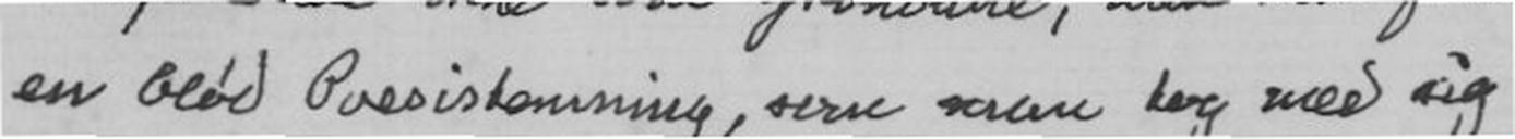en blød Poesistemming, som man tog med sig
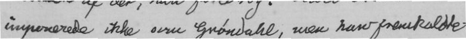imponerede ikke som Grøndahl, men han fremkaldte
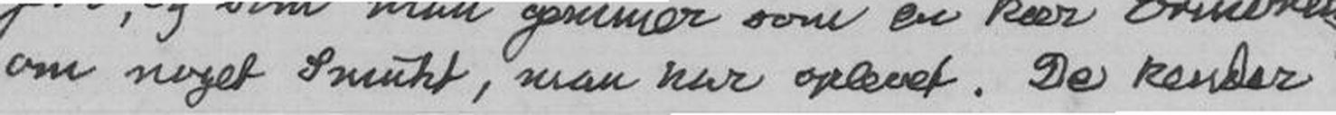om noget Snukt, man har oplevet. De kender
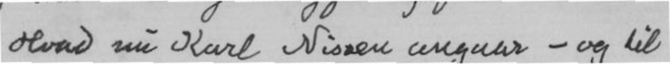Hvad nu Karl Nissen angaar - og til
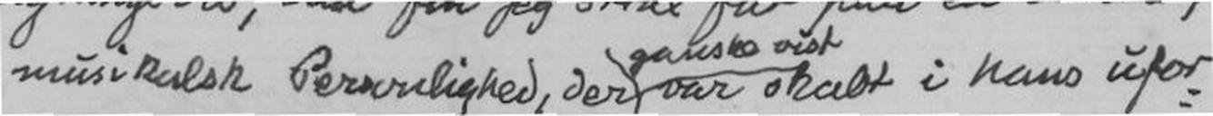musikalsk Personlighed, der var skatt i hans ufor
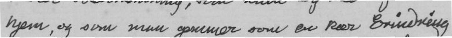hjem, og som maa gemmer som en kær Erindring
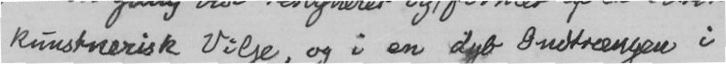kunsnerisk Vilje, og i en dyb Indtrænger i
